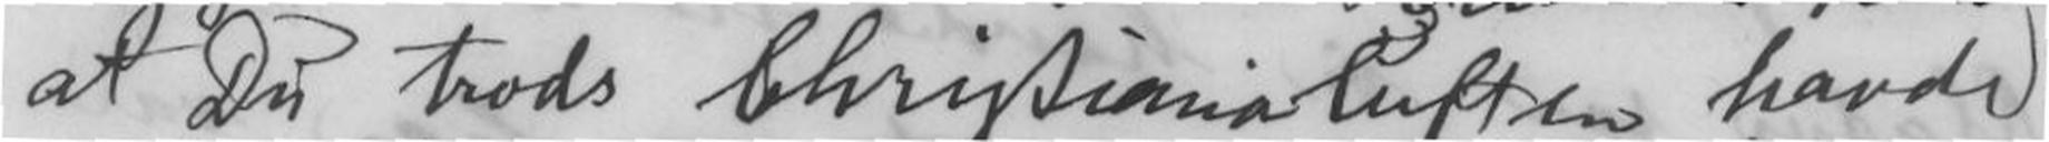at Du trods Christianialuften havde
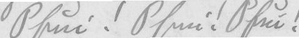Phui! Phui! Phui!
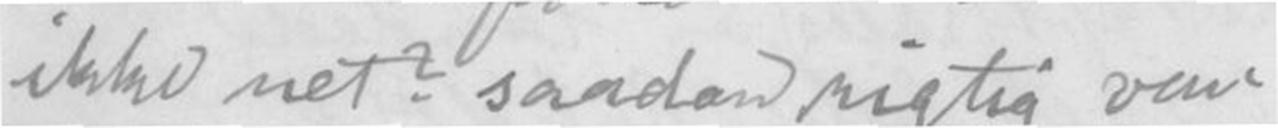ikke ret? saadan rigtig van-
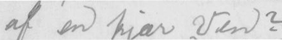af en kjær Ven?
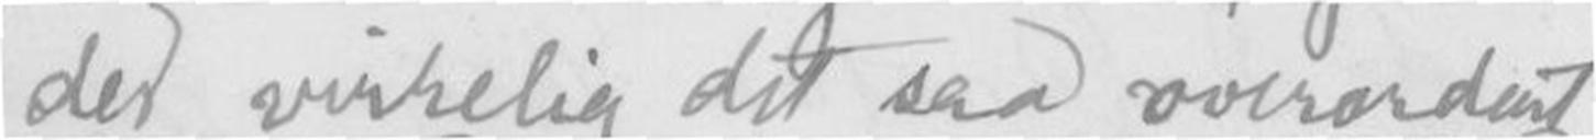des virkelig det saa overordent-
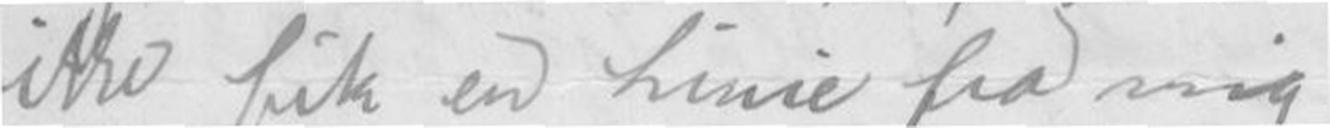ikke fik en Linie fra mig
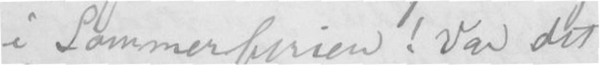i Sommerferien! Var det
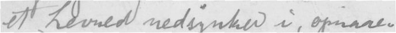et Levned nedsynkede i, opnaae-
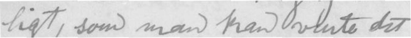ligt, som man kan vente det
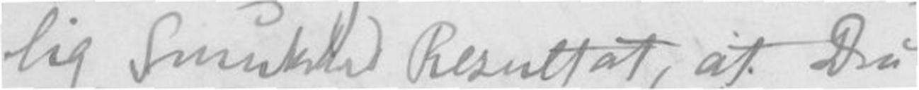lig Smukke Resultat, at Du
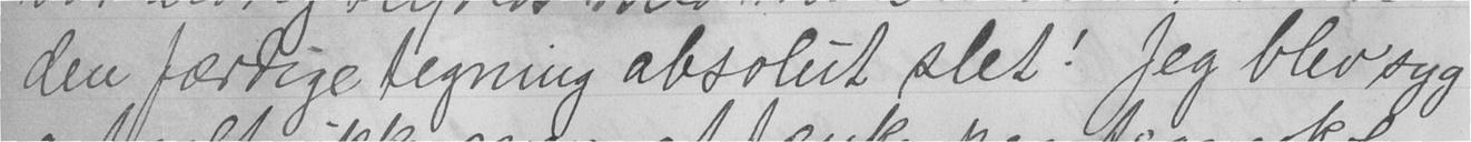den færdige tegning absolut slet! Jeg blev syg
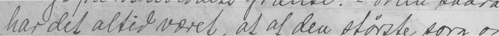har det altid været, at af den største sorg og
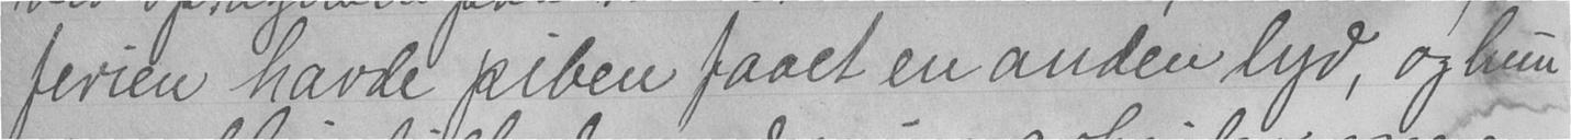ferien havde piben faaet en anden lyd, og hun
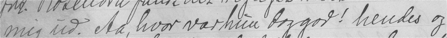mig ud. Aa, hvor var hun dog god! hendes og
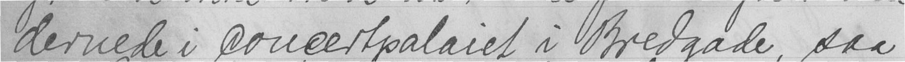dernede i concertpalaiet i Bredgade, saa
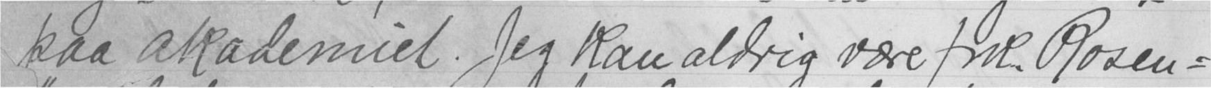paa akademiet. Jeg kan aldrig være frk. Rosen-
